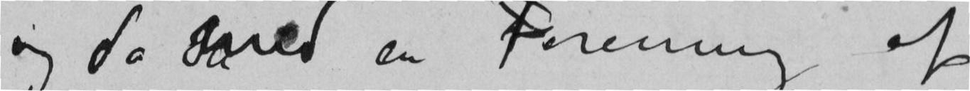og da med en Forening af
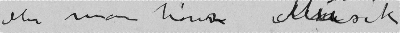eller man hører Musik
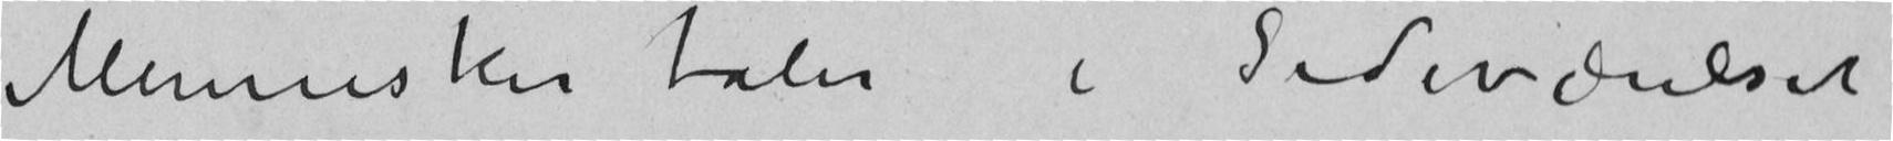Mennesker taler i Sideværelset
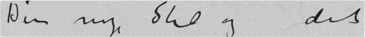din nye Stil og det
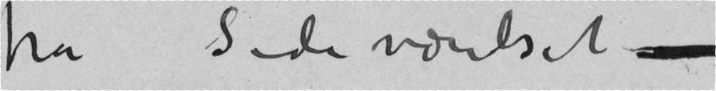fra Sideværelset –
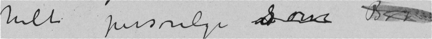helt personlige som B
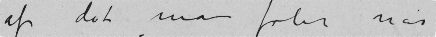af det man føler når
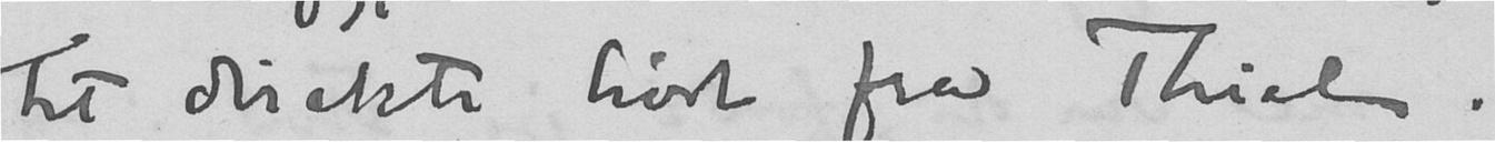tet direkte hørt fra Thiel.
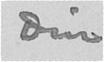Din
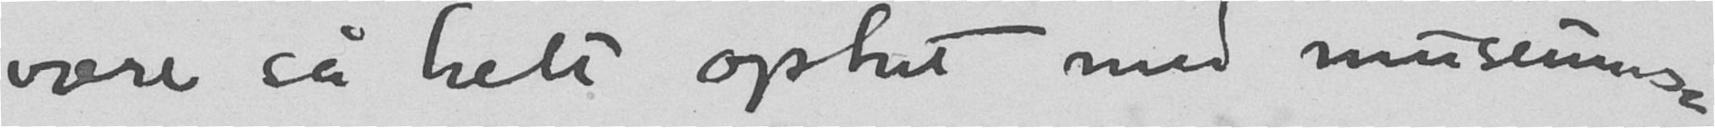være så helt optat med museums-
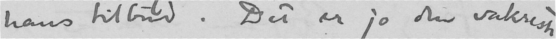hans tilbud. Det er jo den vakreste
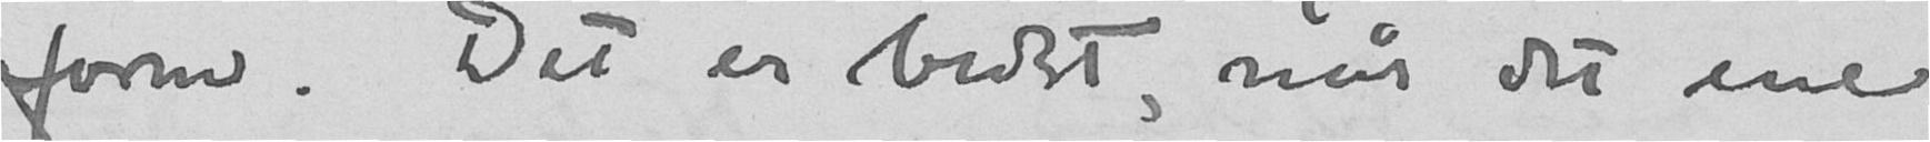form. Det er bedst, når det ene
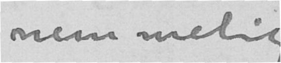nemmelig.
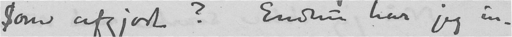som afgjort? Endnu har jeg in-
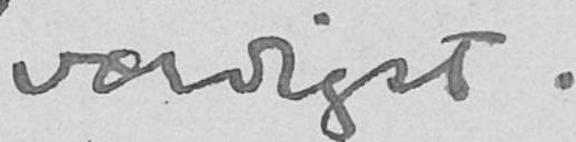værdigst.
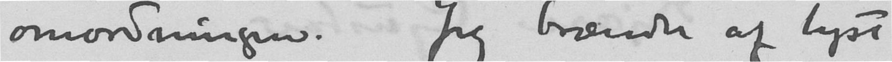omordningen. Jeg brænder af lyst
